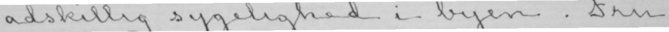adskillig sygelighed i byen. Fru
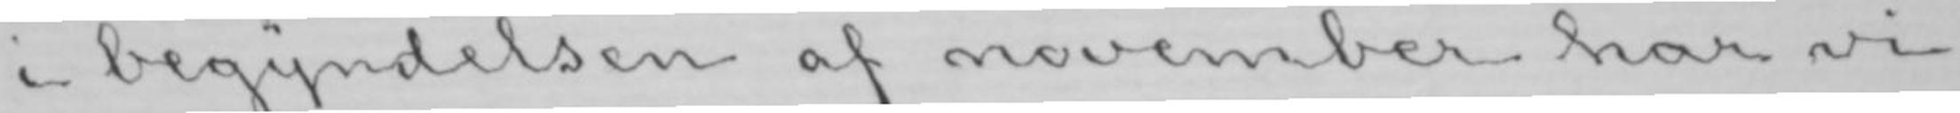i begyndelsen af november har vi
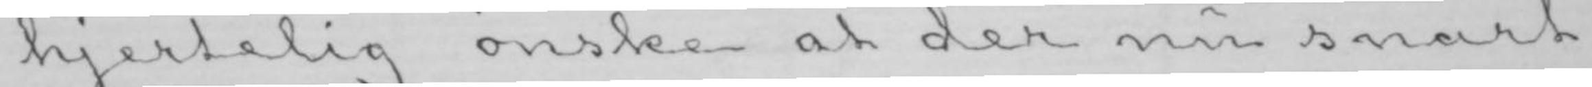hjertelig ønske at der nu snart
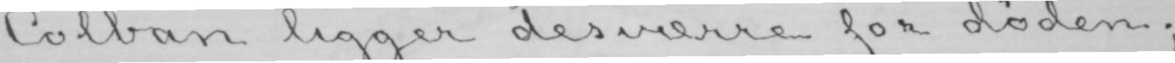Colban ligger desværre for døden;
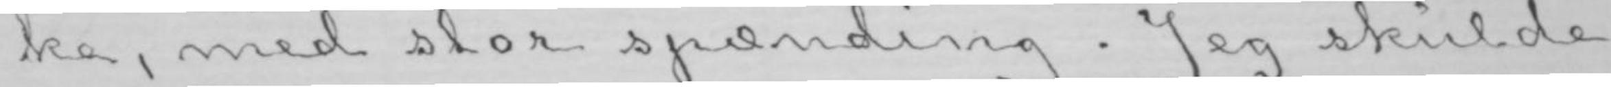ke, med stor spænding. Jeg skulde
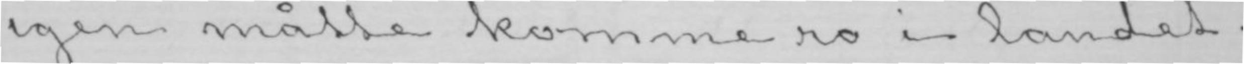igen måtte komme ro i landet.
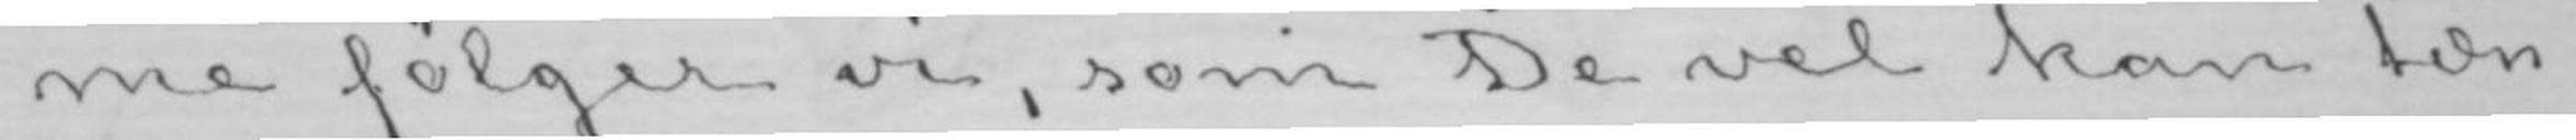me følger vi, som De vel kan tæn-
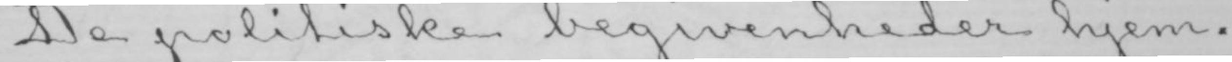De politiske begivenheder hjem-
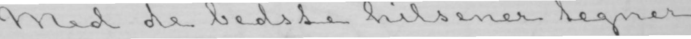Med de bedste hilsener tegner
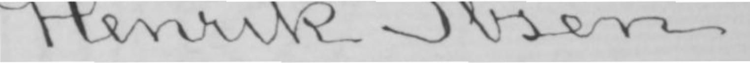Henrik Ibsen.
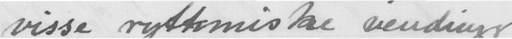visse ryttmiske vendinger
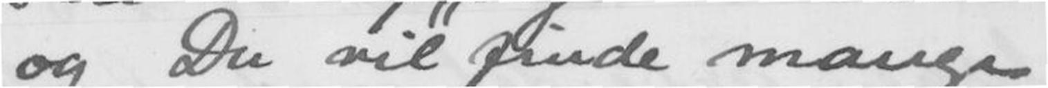og Du vil finde mange
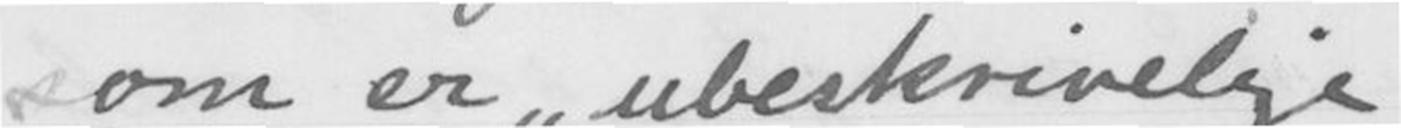som er ubeskrivelige.
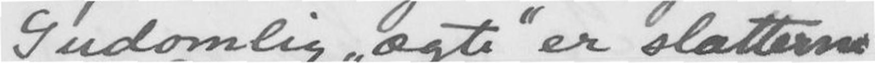Gudomlig ægte er slatterne
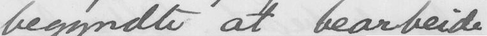begyndte at bearbeide
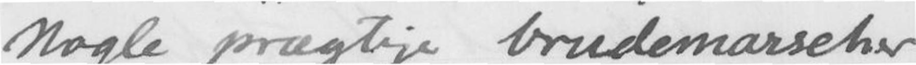Nogle prægtige brudemarscher
